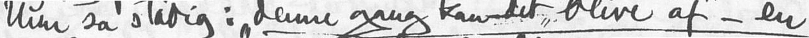Hun sa stadig: "denne gang kan det "blive af' - en
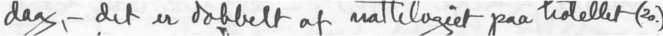dag, - det er dobbelt af nattelogiet paa hotellet (20)
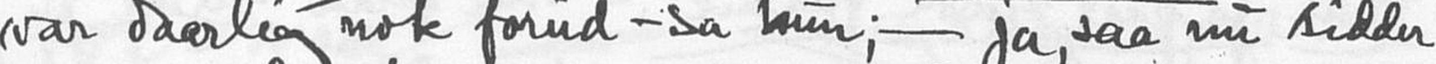var daarlig nok forud - sa hun ; - ja, saa nu sidder
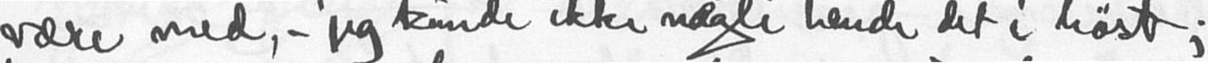være med, - jeg kunde ikke nægte hende det i høst;
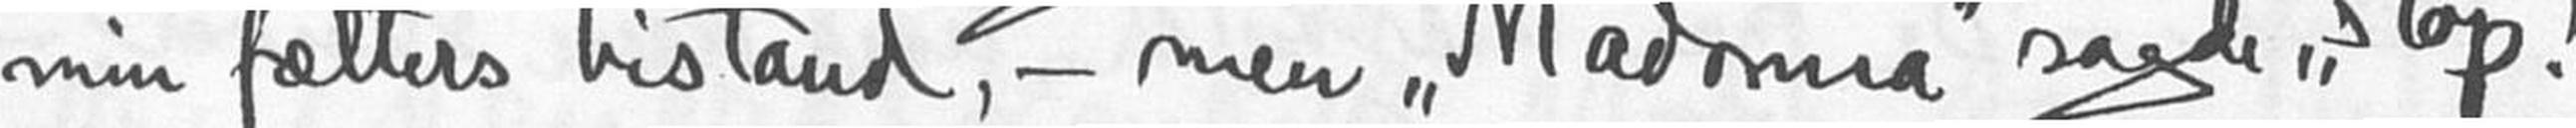min fætters bistand, - men "Madonna" sagde "stop"!
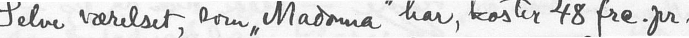Selve værelset, som Madonna har, koster 48 frc. pr.
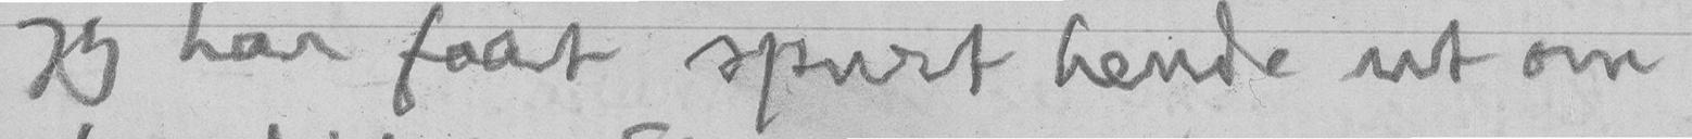jeg har faat spurt hende ut om
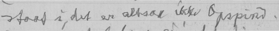staat i, det er altsaa ikke Opspind.
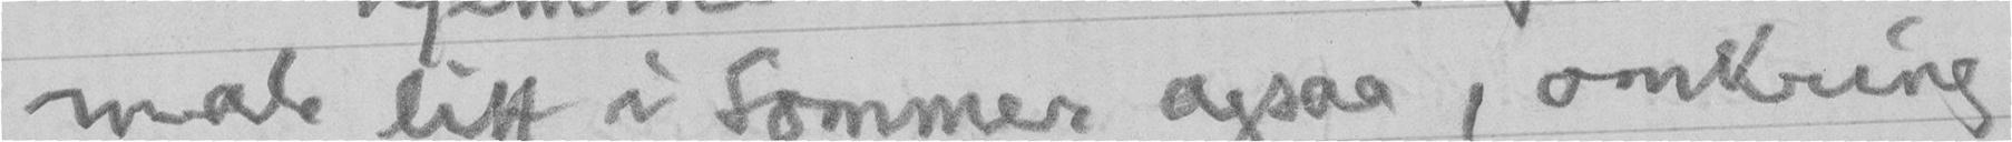male litt i Sommer ogsaa, omkring
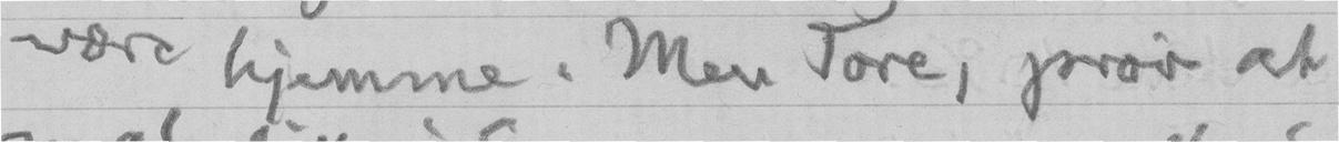være hjemme. Men Tore, prøv at
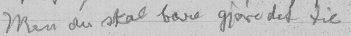Men du skal bare gjøre det til
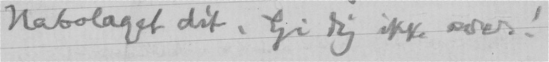Nabolaget dit. Gi dig ikke over!
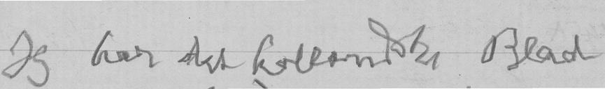Jeg har det hollandske Blad
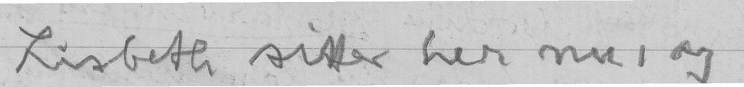Lisbeth sitter her nu, og
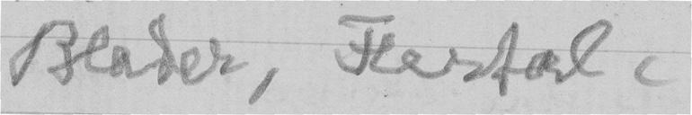Blader, Flertal.
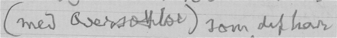(med Oversættelse) som det har
